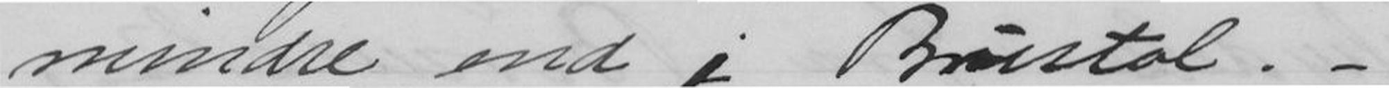mindre end i Bristol. -
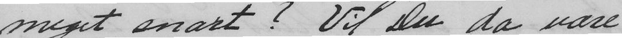meget snart? Vil Du da være
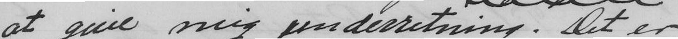at give mig underretning. Det er
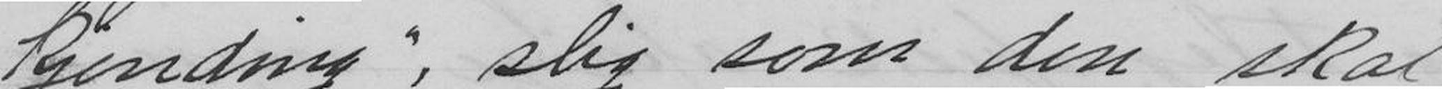kjending", slig som den skal
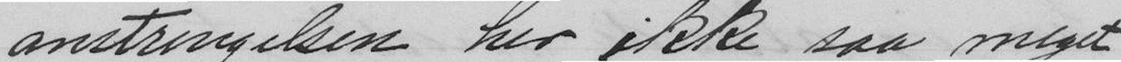anstrengelsen her ikke saa meget
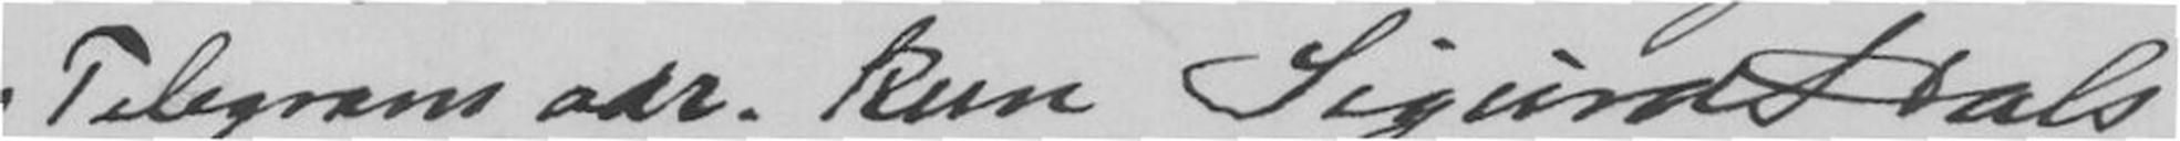Telegram adr. kun Sigurd Hals
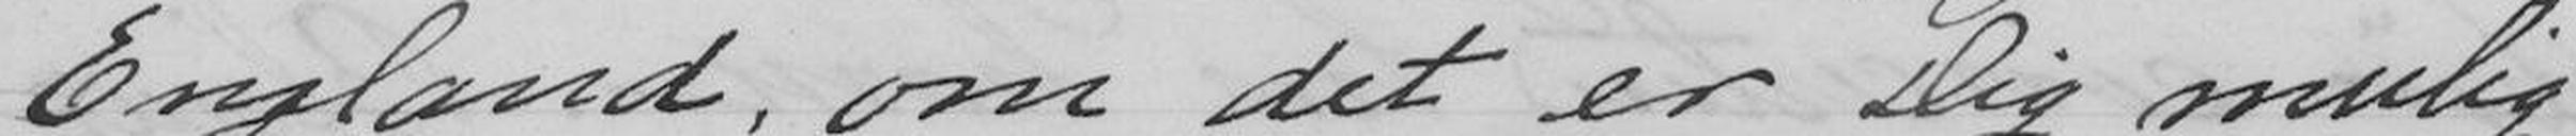England, om det er Dig mulig
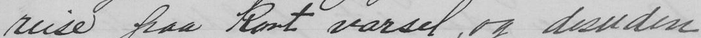reise paa kort varsel, og desuden
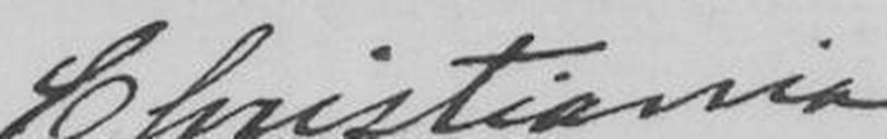Christiania
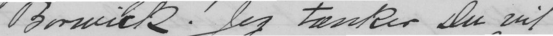Borwick! Jeg tænker Du vil
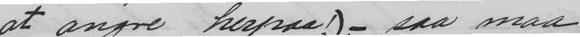at angre herpaa!), - saa maa
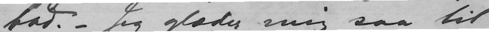bad. - Jeg glæder mig saa til
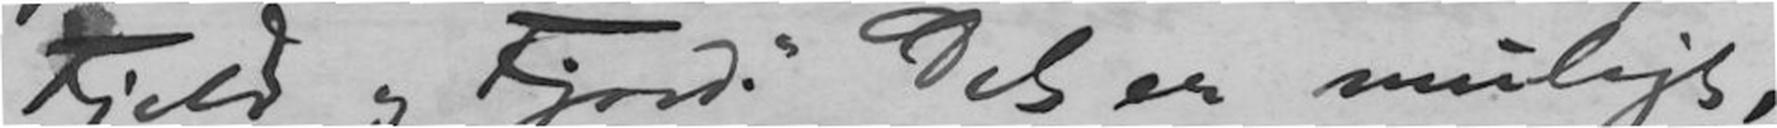Fjeld og Fjord." Det er muligt,
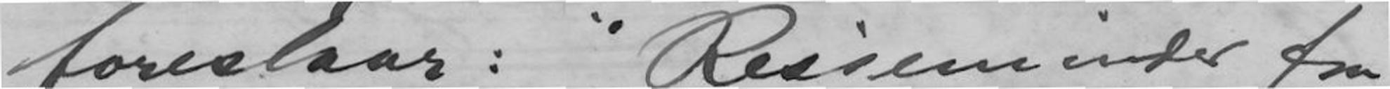foreslaar: "Reiseminder fra
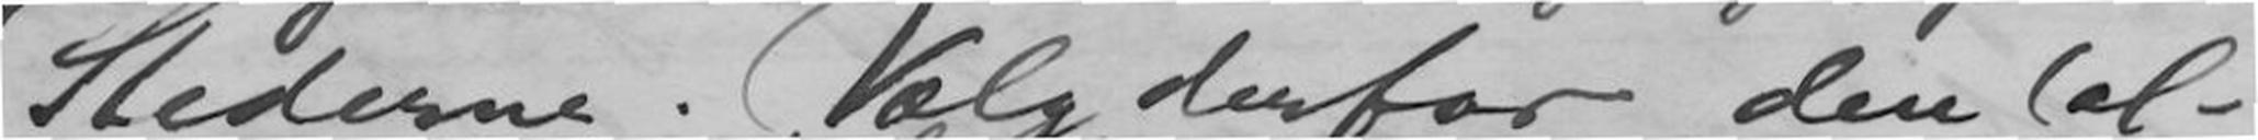Stederne. Vælg derfor den (al-
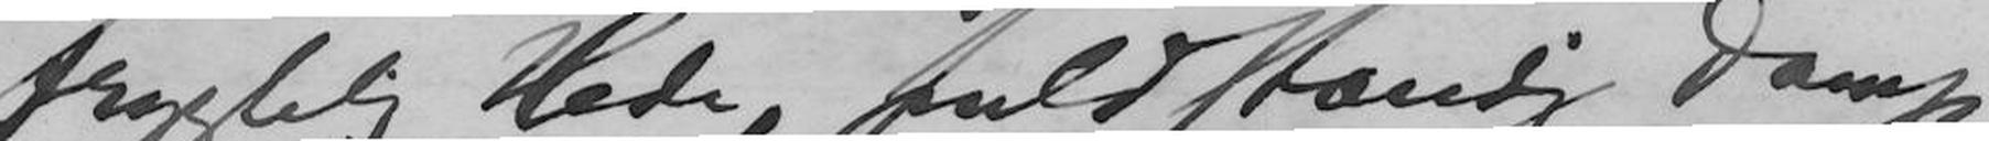frygtelig Hede, fuldstændig Damp-
